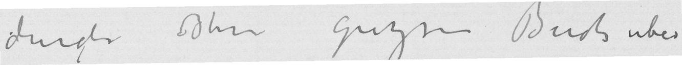durch Ihre grozsen Buch uber
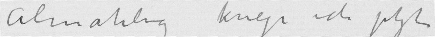Almahlig kriege ich jetzt
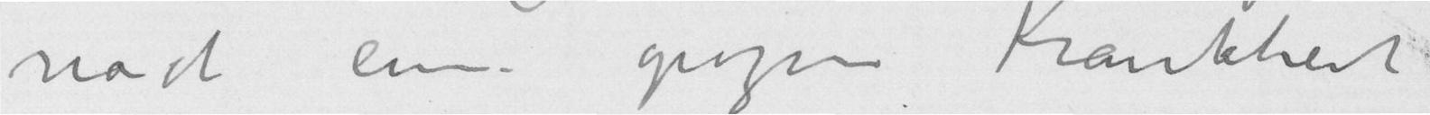nach ein grozsen Krankheit
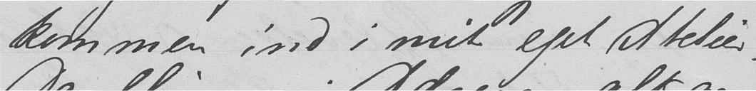kommen ind i mit eget Atelier.
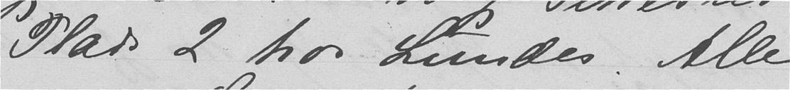Plade J hos Lundes. Alle
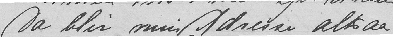Da blir min Adresse altsaa
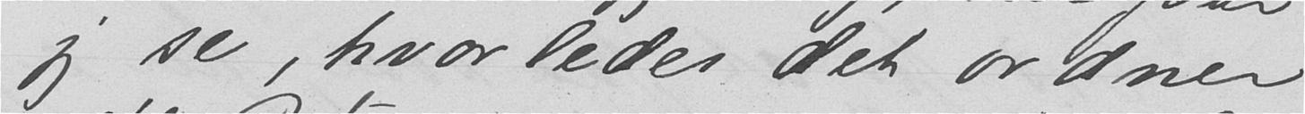jeg se, hvor ledes det ordner
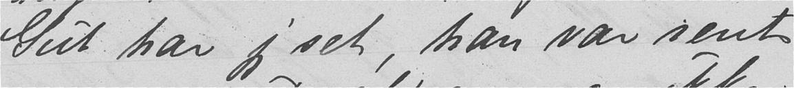Gut har jeg set, han var rent
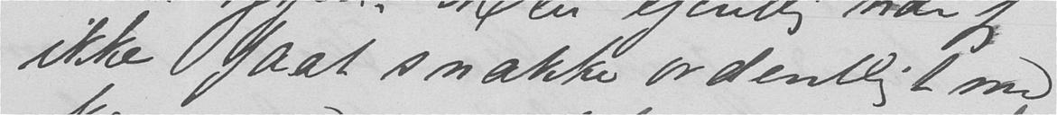ikke faat snakke ordentligt med
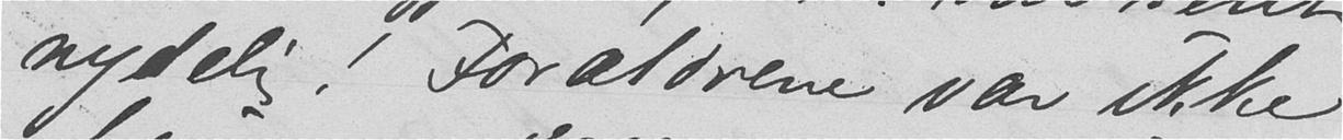nydelig! Forældrene var ikke
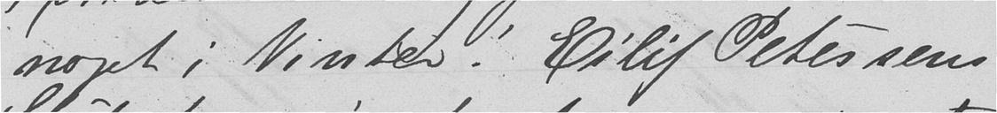noget i Vinter! Eilif Petersens
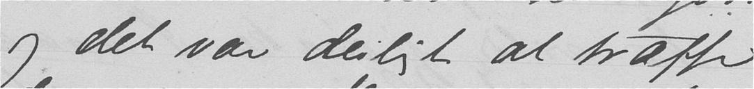og det var deiligt at træffe
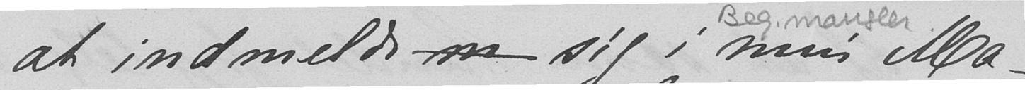at indmelde m sig i min Ma-
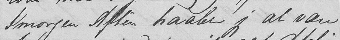Imorgen Aften haaber jeg at være
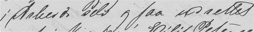i Arbeide selv og faa udrettet
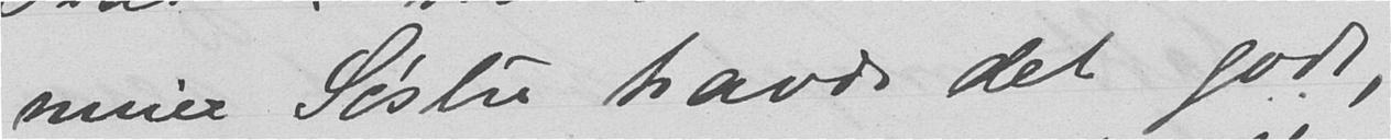mine Søstre havde det godt,
